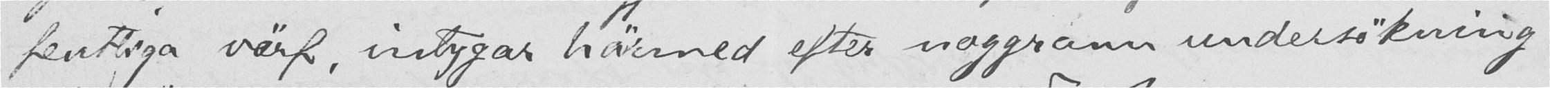fentliga värf, intygar härmed efter noggrann undersøkning
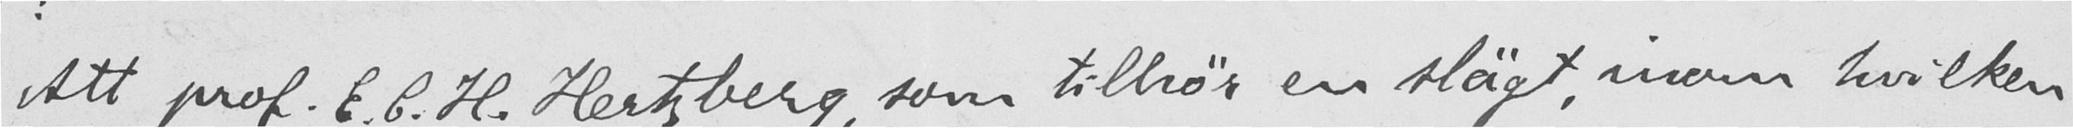Att prof. E. C. H. Hertzberg, som tilhør en slägt, inom hvilken
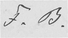F. B.
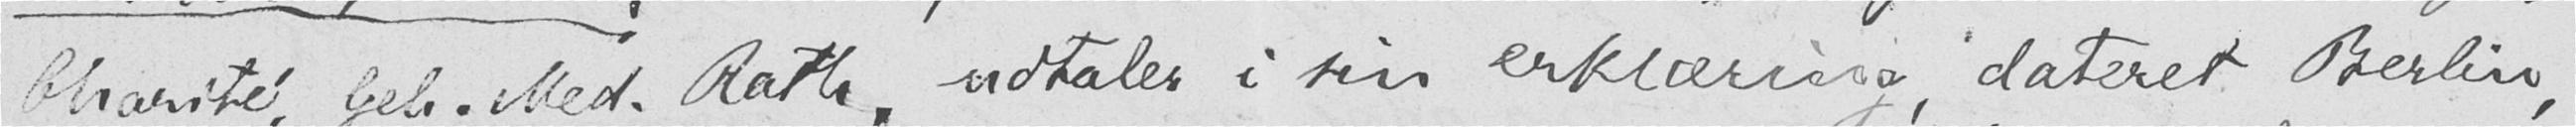Charité. Geh. Med. Rath, udtaler i sin erklæring dateret Berlin,
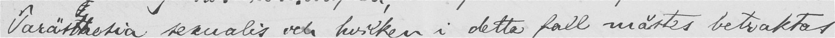Parästhesia sexualis, och hvilken i dette fall måstes betraktas
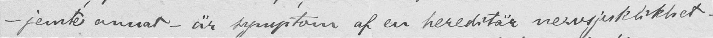– jemte annat – är symptom af en hereditär nervsjuklikhet - -
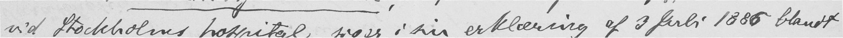vid Stockholms hospital, siger i sin erklæring af 3 juli 1886 blandt
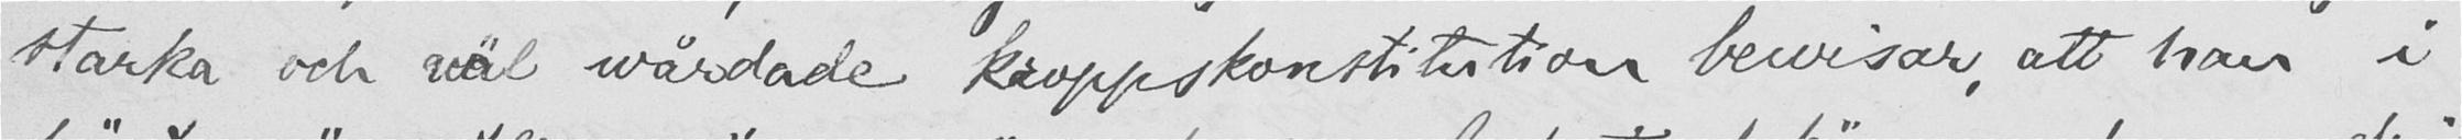starka och väl wårdade kroppskonstitution bevisar, att han i
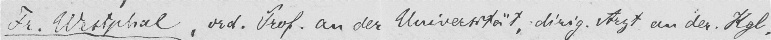Fr. Westphal, ord. Prof. an der Universität, dirig. Argt an der. Kgl.
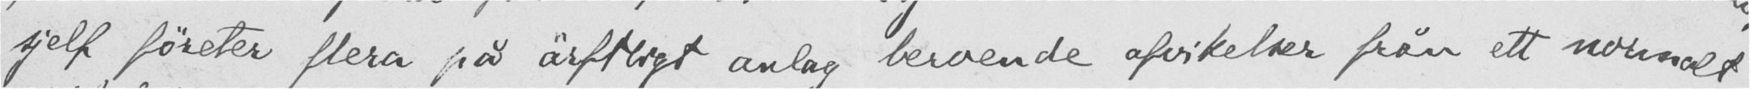sjelf føreter flera på ärftligt anlag beroende afvikelser från ett normalt
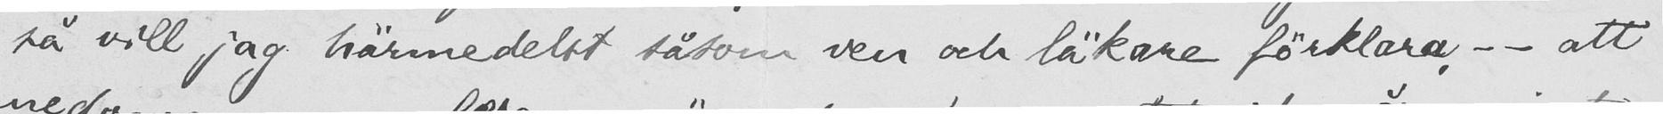- - så vill jag härmedelst såsom ven och läkare førklara, - - att
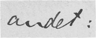andet:
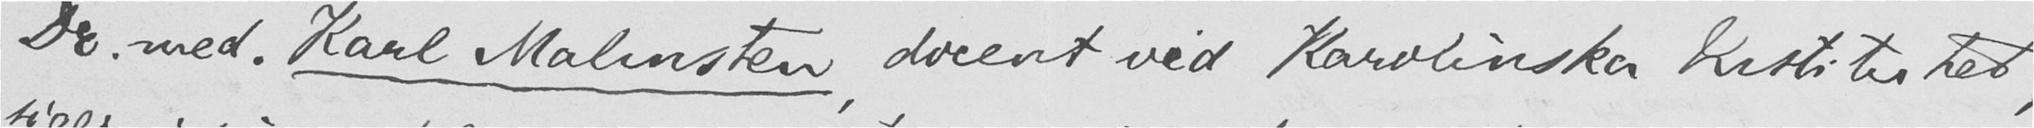Dr. med. Karl Malmsten, docent ved Karolinska Institutet,
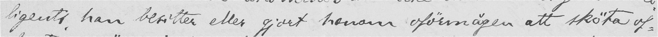ligents han besitter eller gjort honom oførmågen att skøta of-
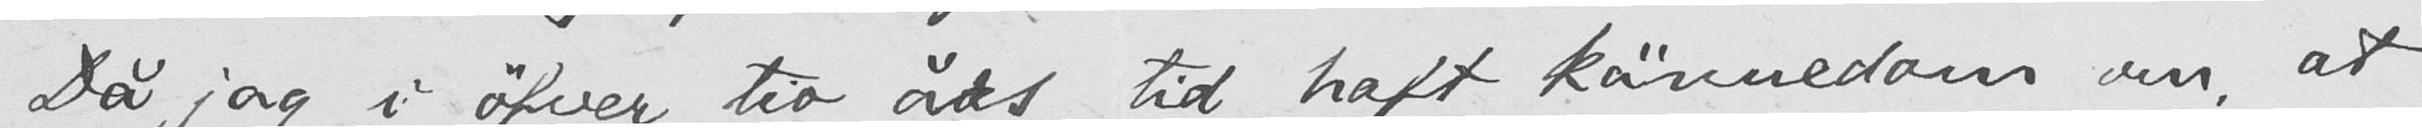Då jag i øfver tio års tid haft kännedom om, at
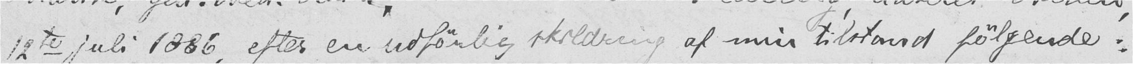12te juli 1886, efter en udførlig skildring af min tilstand følgende:
